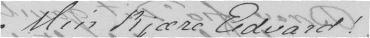Min kjære Edvard!
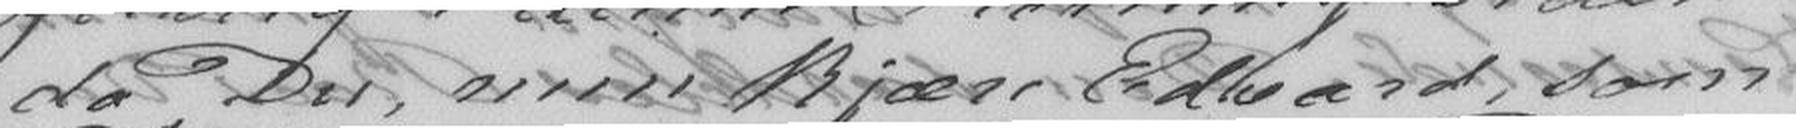da Du, min kjære Edvard, som
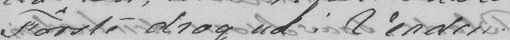Første drog ud i verden.
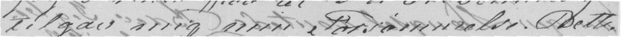tilgav mig min Forsømmelse. Bette-
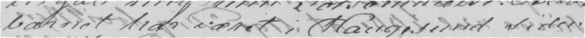barnet har været i Haugesund siden
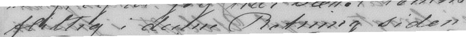flittig i denne Retning siden
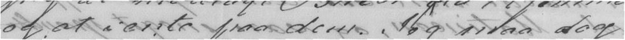og at vænte paa dem. Jeg maa dog
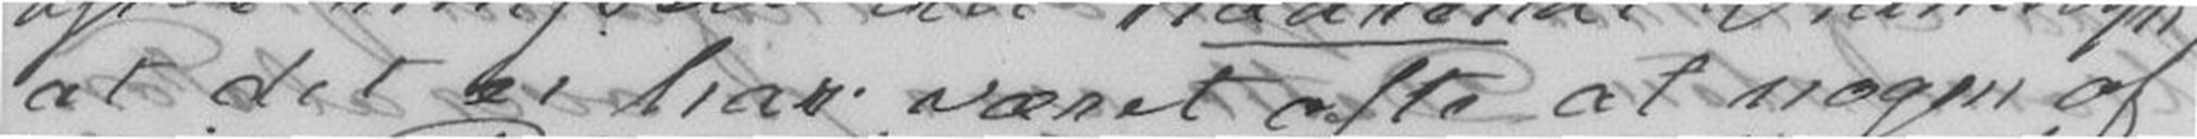at det ei har været ofte at nogen af
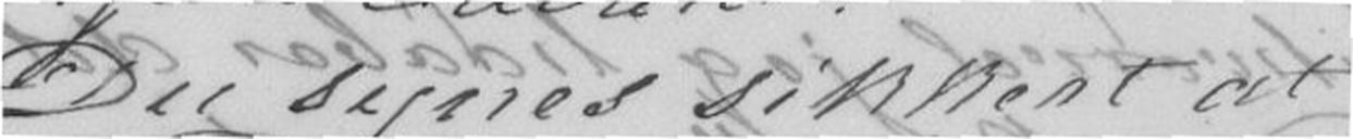Du synes sikkert at
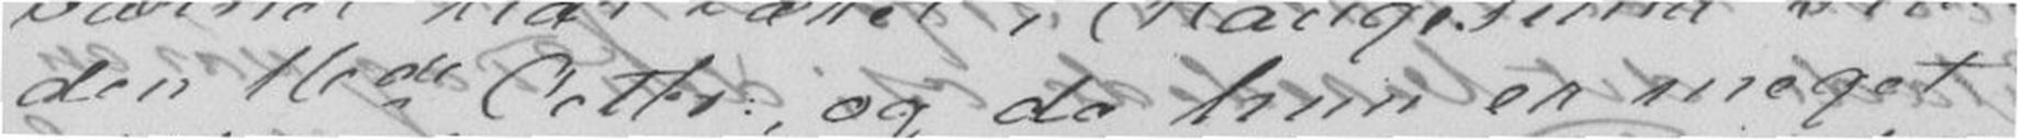den 16de Octbr, og da hun er meget
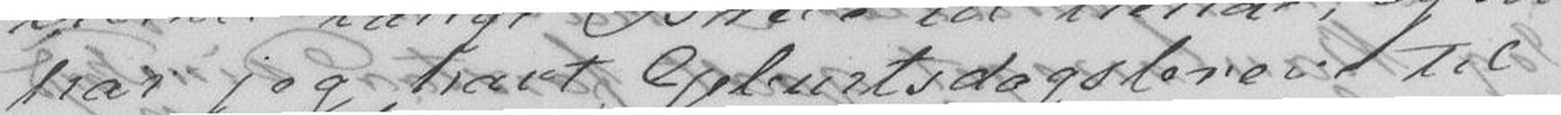har jeg havt Geburtsdagsbrev til
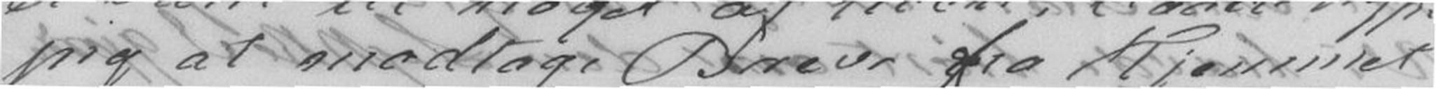pig at modtage Brev fra Hjemmet
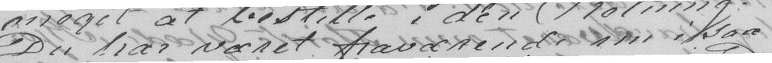Du har været fraværende nu i saa
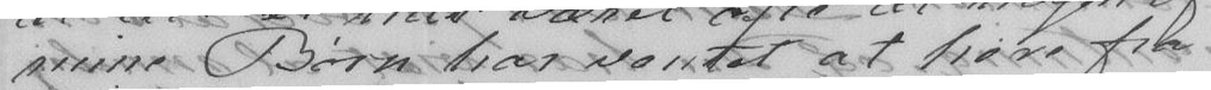mine Børn har ventet at høre fra
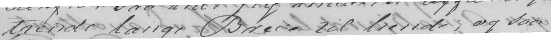trende lange Breve til hende, og saa
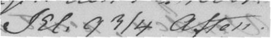Kl. 9 3/4 Aften.
